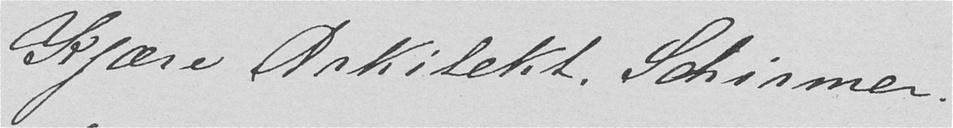Kjære Arkitekt. Schirmer.
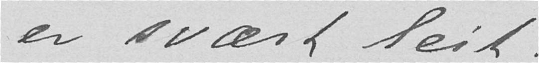er svært leit.
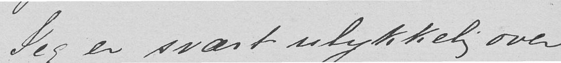Jeg er svært ulykkelig over
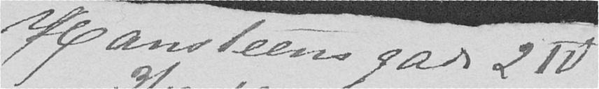Hansteens gade 2 IV
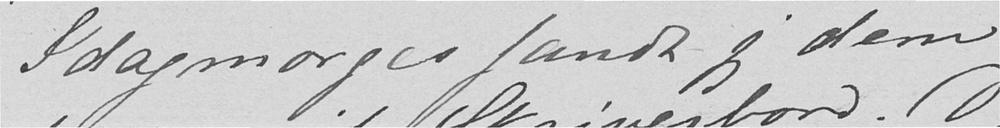Idagmorges fandt jeg dem
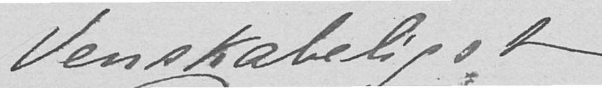Venskabeligst
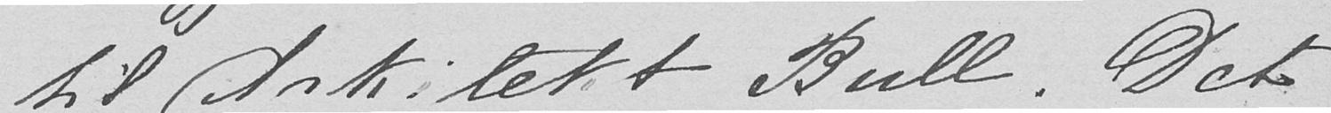til Arkitekt Bull. Det
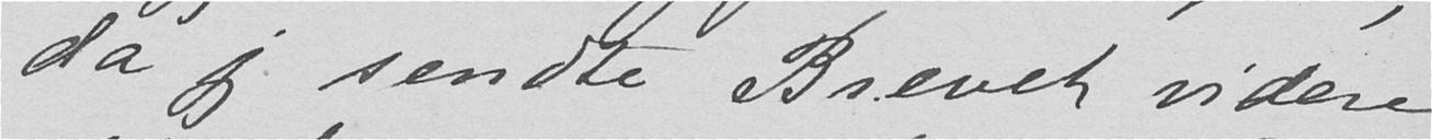da jeg sendte Brevet videre
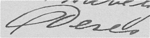Deres
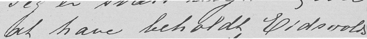at have beholdt Eidsvolds
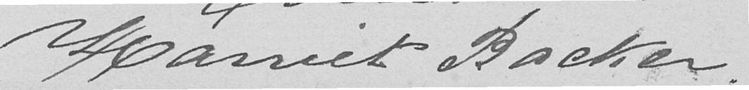Harriet Backer.
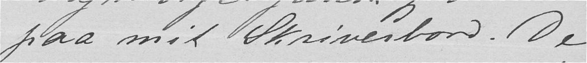paa mit Skriverbord. De
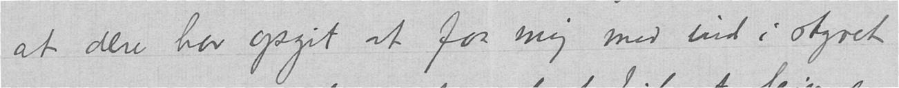at dere har opgit at faa mig med ind i styret
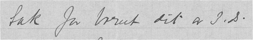tak for brevet dit av 3.ds.
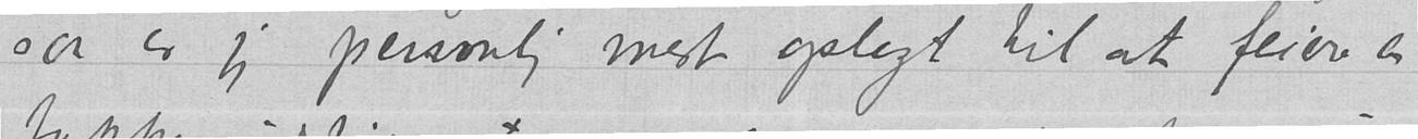saa er jeg personlig mest oplagt til at feiere en
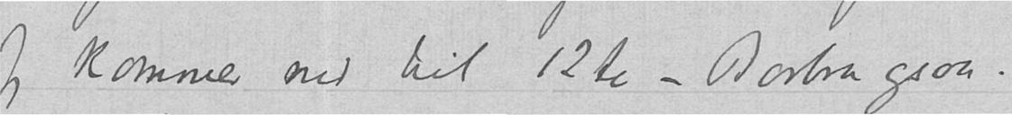Jeg kommer ned til 12te – Barbra ogsaa.
